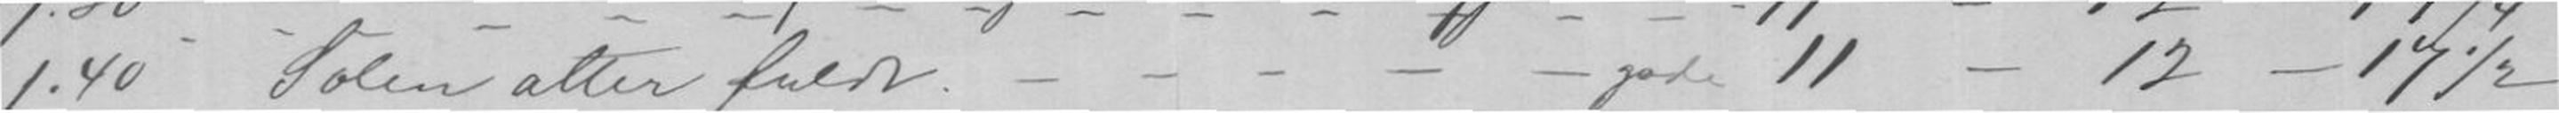" 1.40 Solen atter fuldt _ _ _ _ _ _ _ _ _ _ _ 11 - 12 - 17½
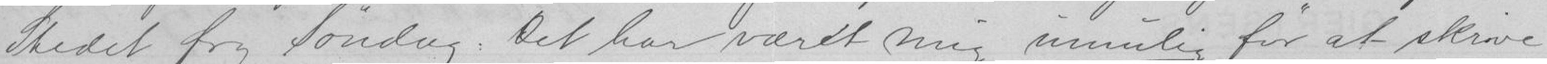Stedet frg Søndag. Det har været mig umulig før at skrive
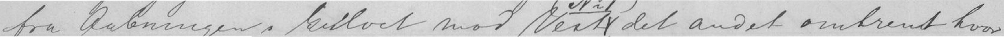fra Aabningen i Gulvet mod Vest, det andet omtrent hvor
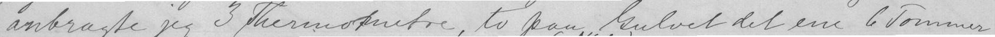anbragte jeg 3 Thermometre, to paa Gulvet det ene 6 Tommer
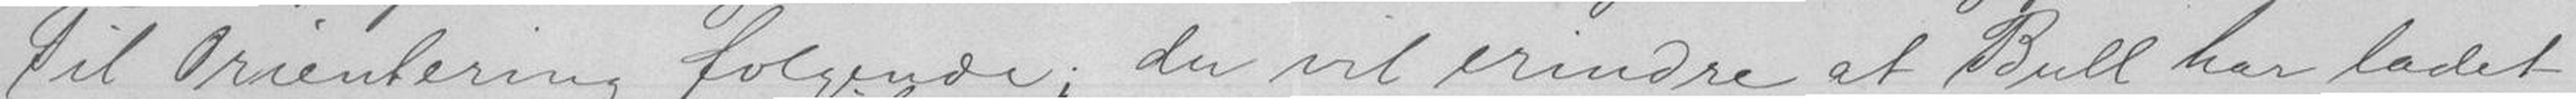Til Orientering følgende du vil erindre at Bull har ladet
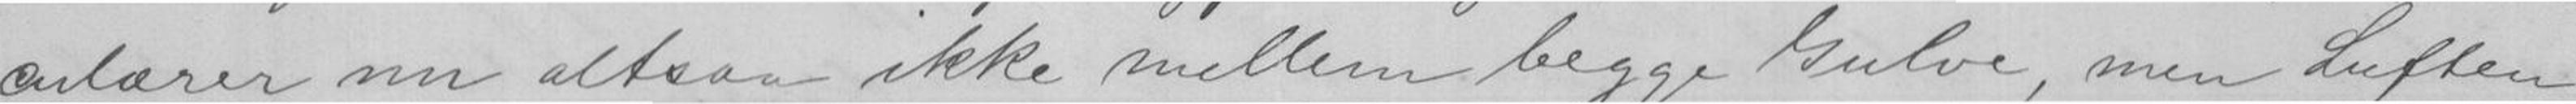culærer nu altsaa ikke mellem begge Gulve, men Luften
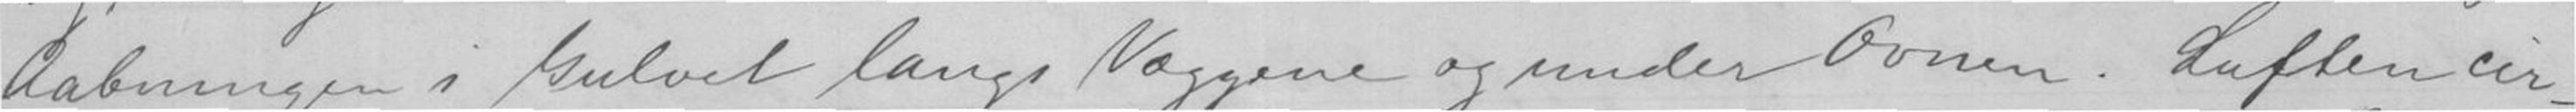Aabningen i Gulvet langs Væggene og under Ovnen. Luften cir-
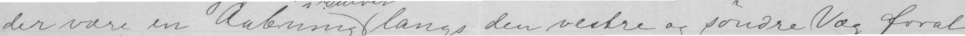der være en Aabning langs den vestre og søndre Væg forat
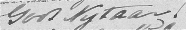Godt Nytaar!
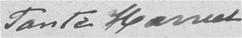Tante Harriet
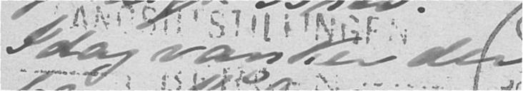Idag vanker der
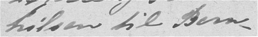hilsen til Bern-
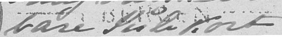bare Julekort
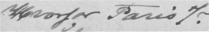Hvorfor Paris 7?
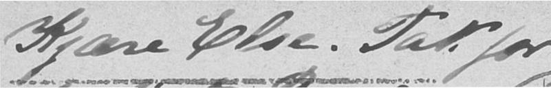Kjære Else. Tak for
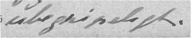ubegripeligt.
